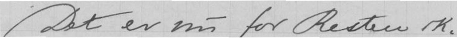Det er nu for Resten ik-
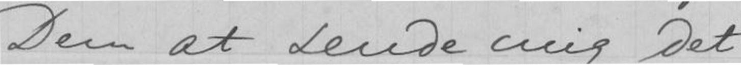Dem at sende mig det
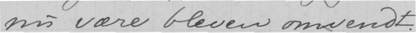nu være bleven omvendt.
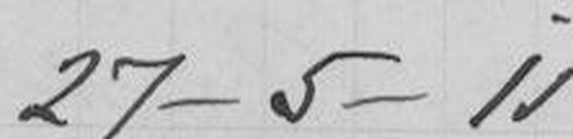27-5-11
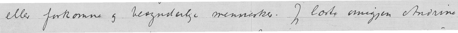eller forkomne og besynderlige mennesker. Jeg læste omigjen Andrine
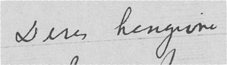Deres hengivne
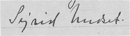Sigrid Undset.
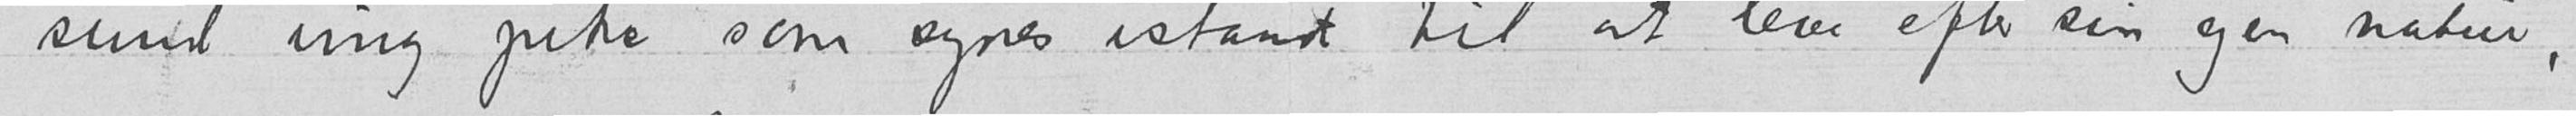sund ung pike som synes istand til at leve efter sin egen natur,
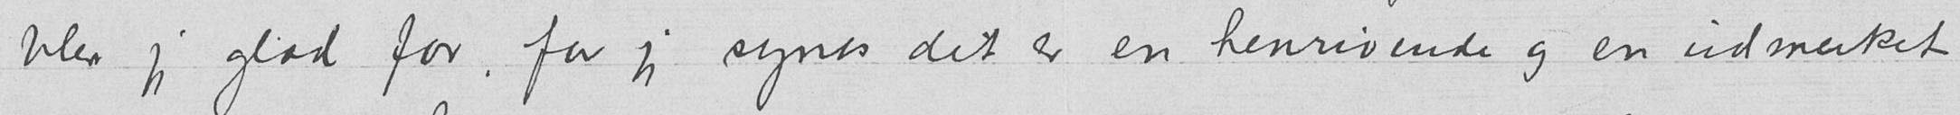blev jeg glad for, for jeg synes det er en henrivende og en udmerket
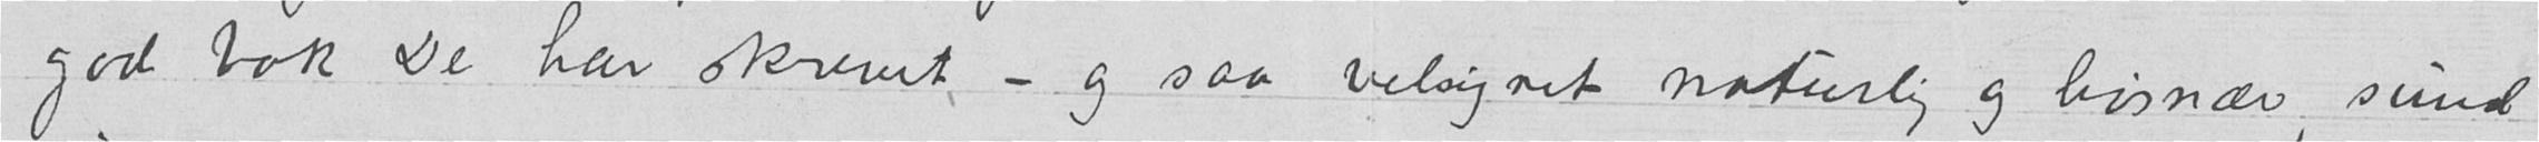god bok De har skrevet – og saa velsignet naturlig og livsnær, sund
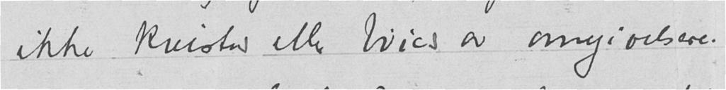ikke kviste eller bøies av omgivelsene.
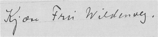Kjære Fru Wildenvey,
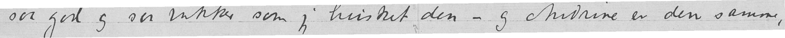saa god og saa vakker som jeg husket den – og Andrine er den samme,
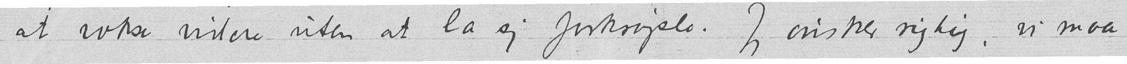at vokse videre uten at la sig forkrøple. Jeg ønsker rigtig, vi maa
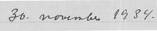30. november 1934.
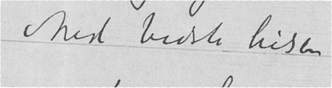Med bedste hilsen
